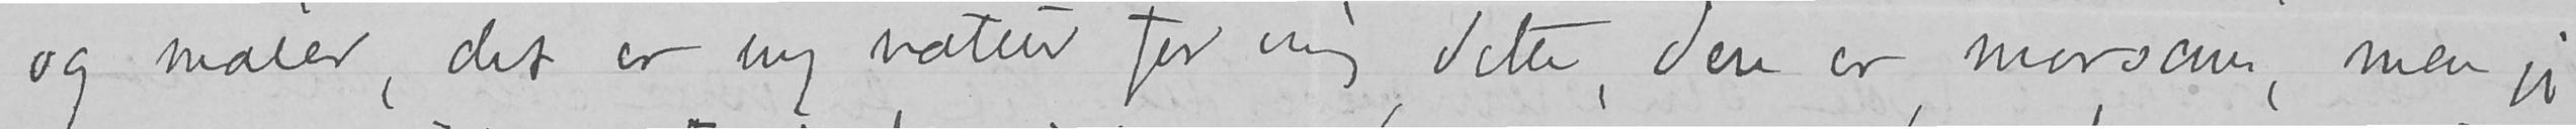og maler, det er ny natur for mig dette, den er morsom, men jeg
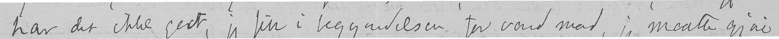har det ikke godt, jeg fik i begyndelsen for vond mad, jeg maatte gjøre
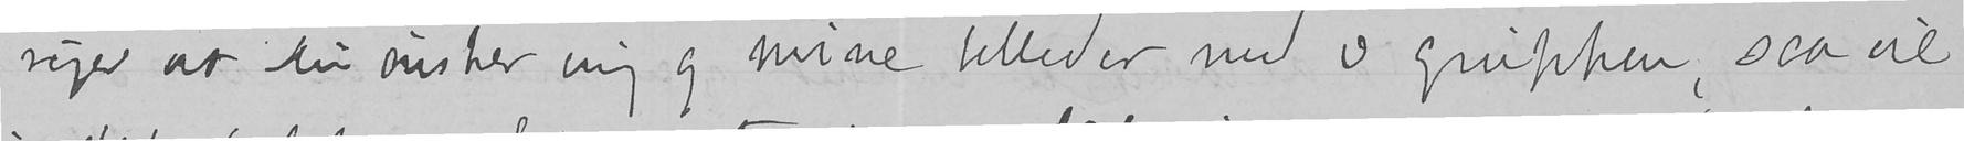siger at Du ønsker mig og mine billeder med i gruppen, saa vil
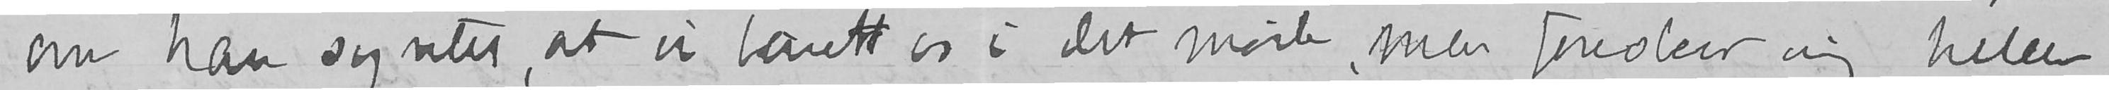om han syntes, at vi barett os i det møde, men foreslaar mig heller
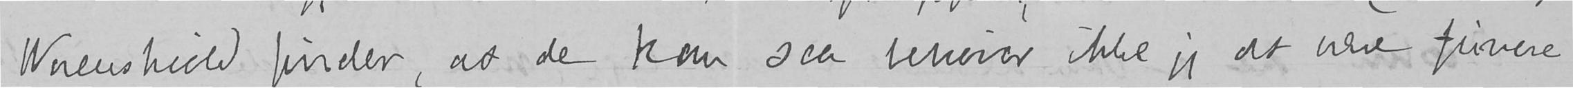Werenskiold finder, at de kan saa behøver ikke jeg at være finere
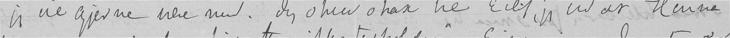jeg vil gjerne være med. Jeg skrev strax til Eilif, jeg ved at Hanna
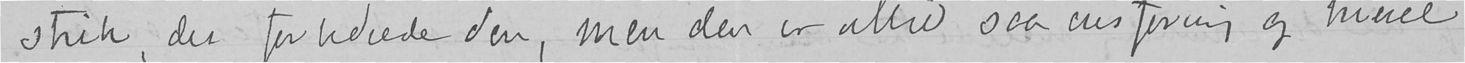strik, det forbedrede den, men den er altid saa ensformig og triveel
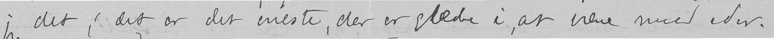jeg det; det er det eneste, der er glæde i, at være med eder.
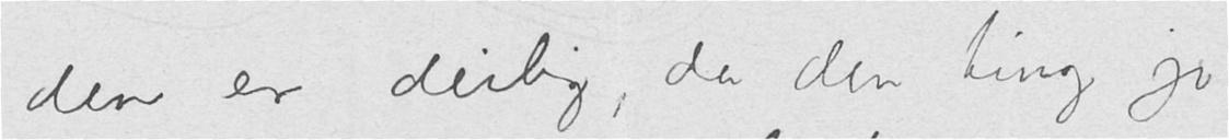den er deilig, da den ting jo
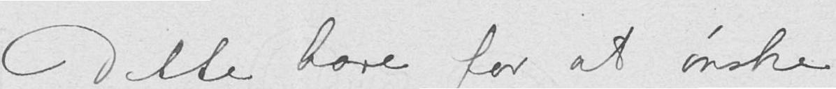Dette bare for at ønske
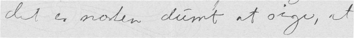det er nesten dumt at sige, at
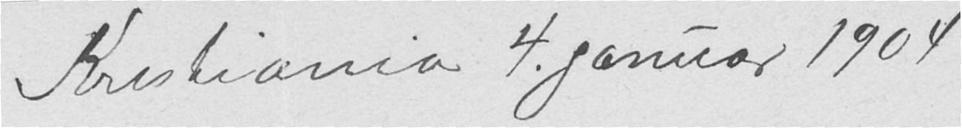Kristiania 4. januar 1904
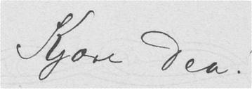Kjære Dea!
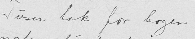Tusen tak for bogen —
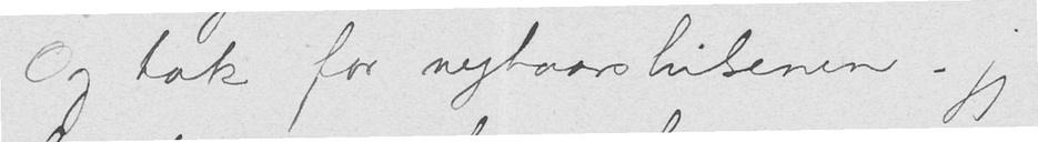Og tak for nytaarshilsenen — jeg
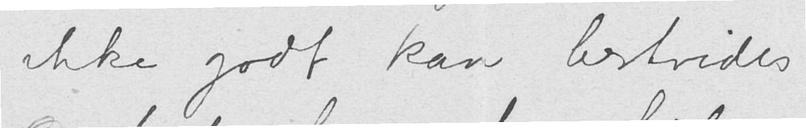ikke godt kan bestrides.
